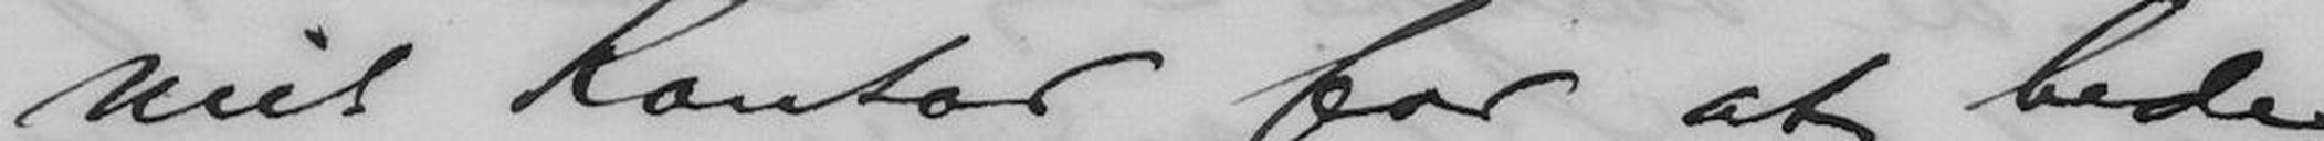mit Kontor for at bede
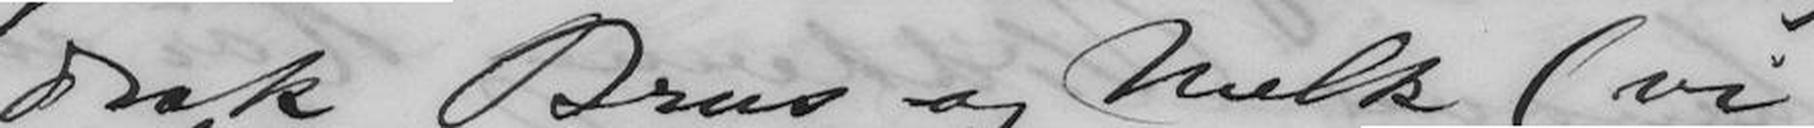drak Brus og Melk (vi
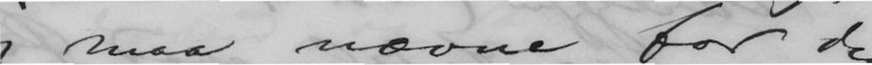jeg maa nævne for Dig,
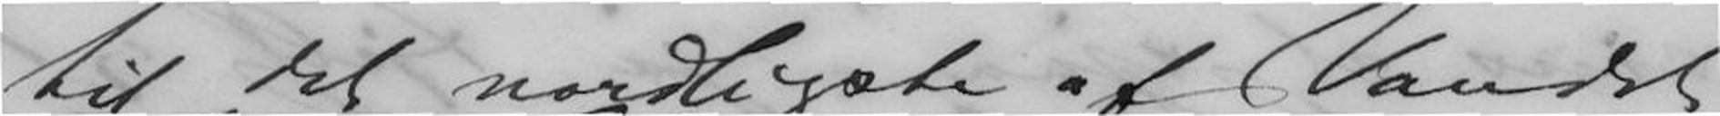til det nordligste af Vandet
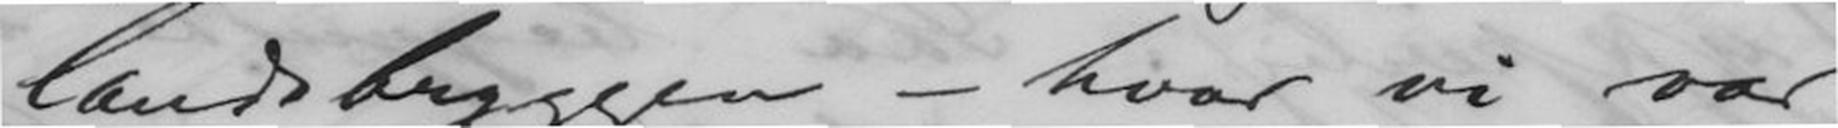landsbryggen - hvor vi var
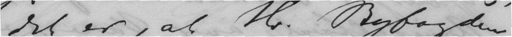det er, at Hr. Byfogden
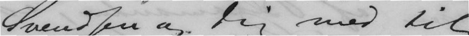Svendsen og Dig med til
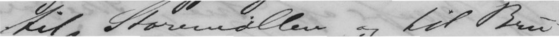til Stormøllen og til Bru-
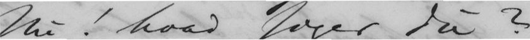Nu! hvad siger Du?
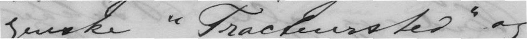genske "Tracteursted" og
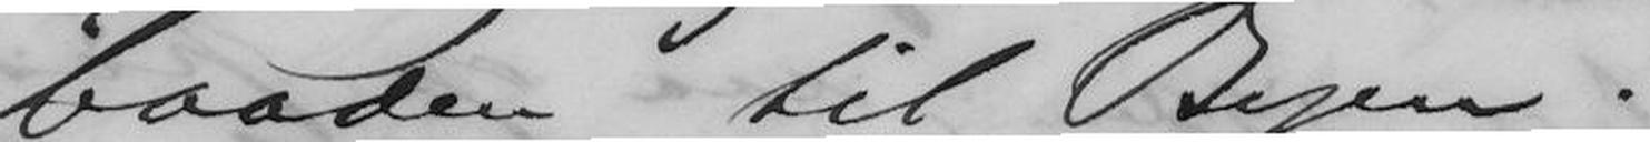baaden til Bergen!
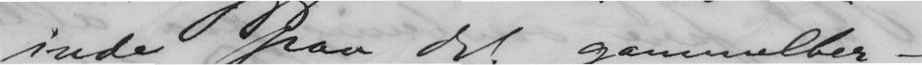inde paa det gammelber-
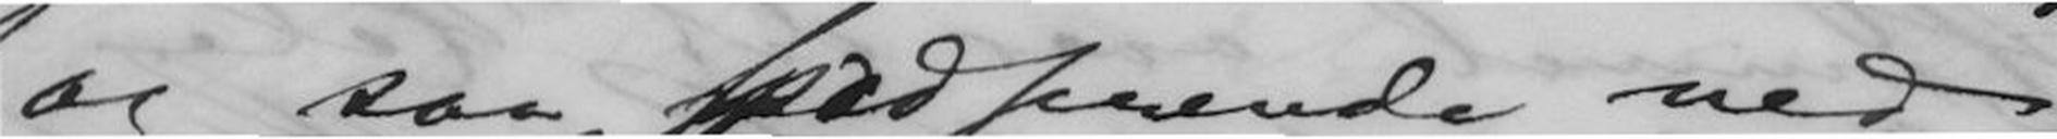og saa spadserende ned
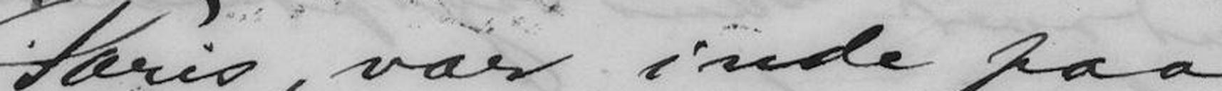Paris, var inde paa
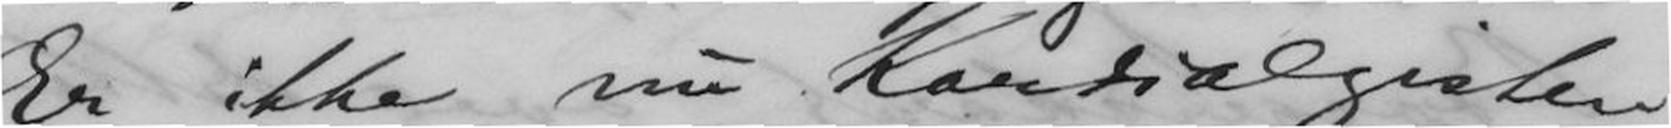Er ikke nu Kardialgisten
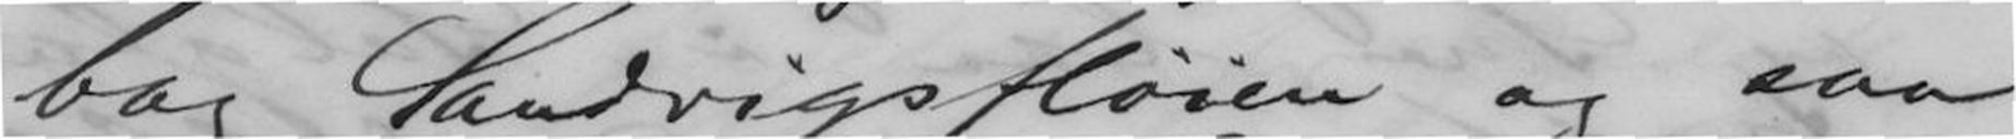bag Sandvigsfløien og saa
